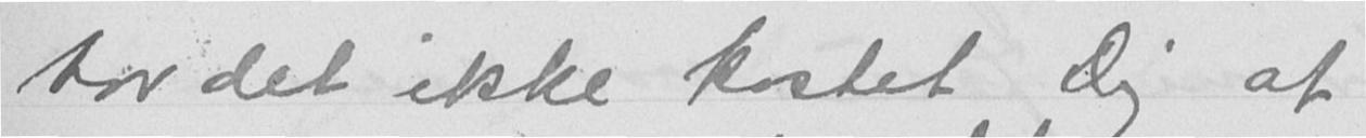har det ikke kostet Dig at
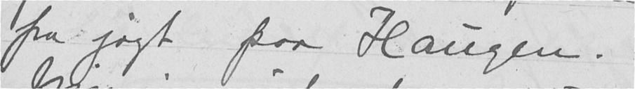fra jagt paa Haugen.
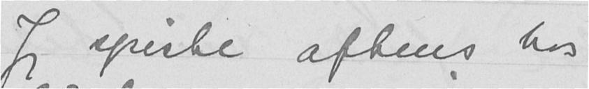Jeg spiste aftens hos
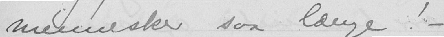mennesker saa længe! -
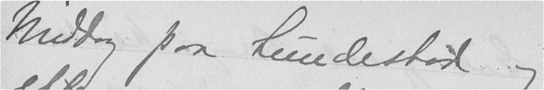Middag paa Lundestad og
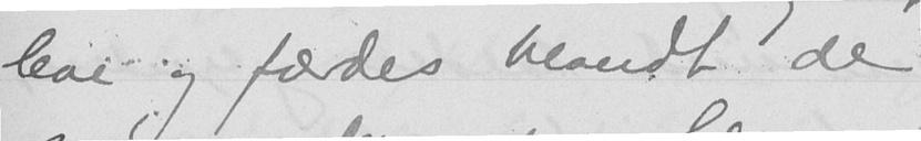leve og færdes blandt de
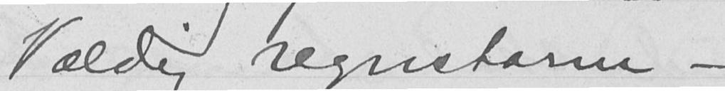Vældig regnstorm -
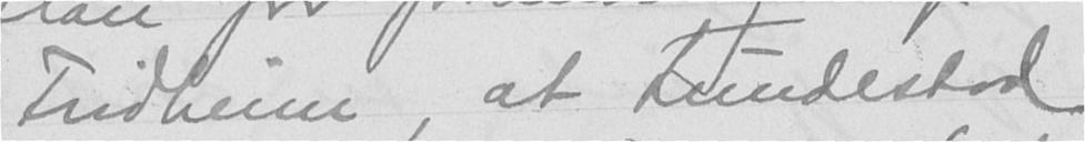Fridheim, at Lundestad
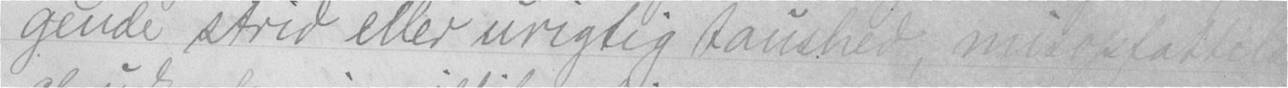gende strid eller urigtig taushed, misopfattelse
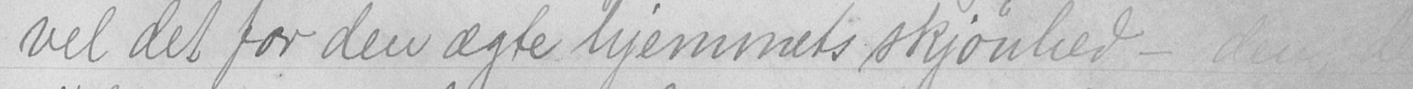vel det for den ægte hjemmets skjønhed - den der
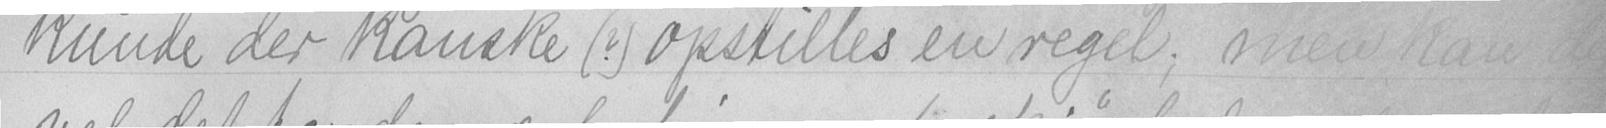kunde der kanske (?) opstilles en regel; men kan der
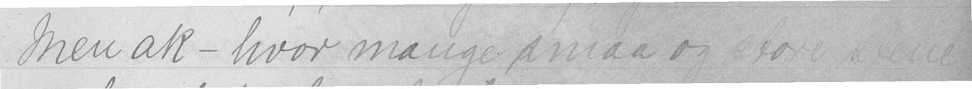Men ak - hvor mange smaa og store stene er
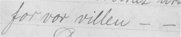for vor villen - -
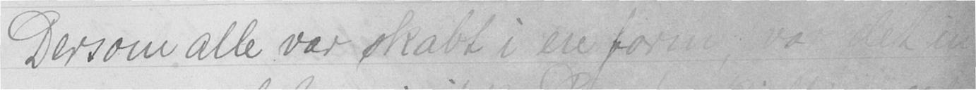Dersom alle var skabt i en form, var det in-
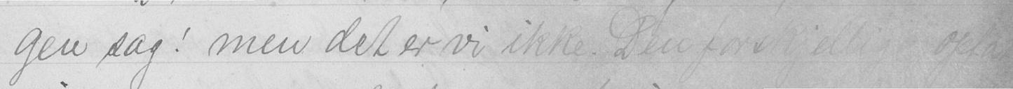gen sag! men det er vi ikke. Den forskjellige opfar-
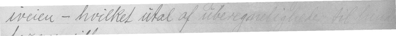iveien - hvilket utal af uberegneligheder til hinder
